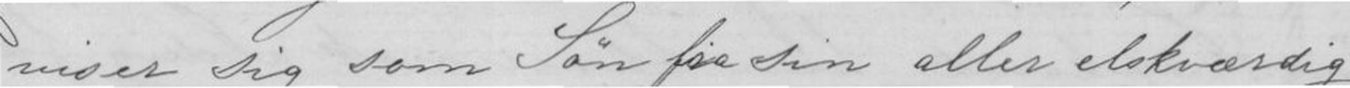viser sig som Søn for sin aller elskværdig-
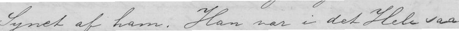Synet af ham. Han var i det Hele saa
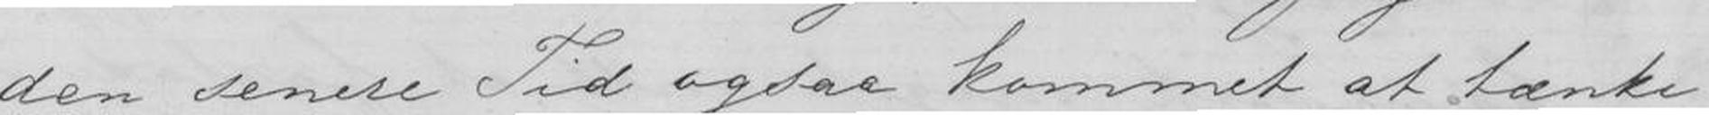den senere Tid ogsaa kommet at tænke
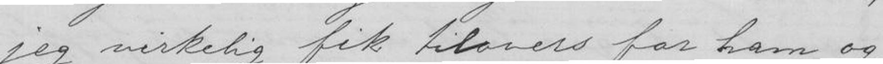jeg virkelig fik tilovers for ham og
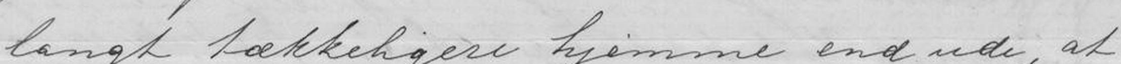langt tækkeligere hjemme end ude, at
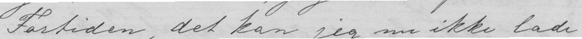Fortiden, det kan jeg nu ikke lade
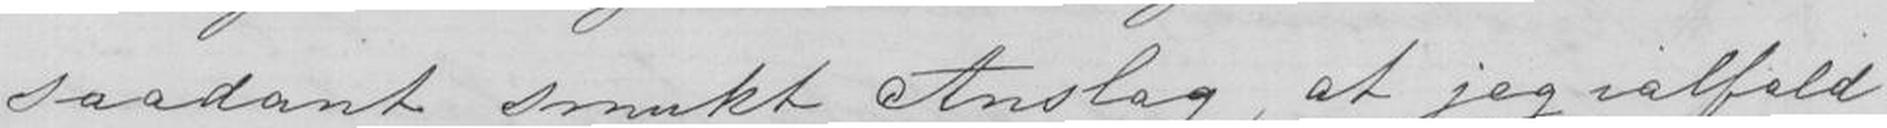saadant smukt Anslag, at jeg ialfald
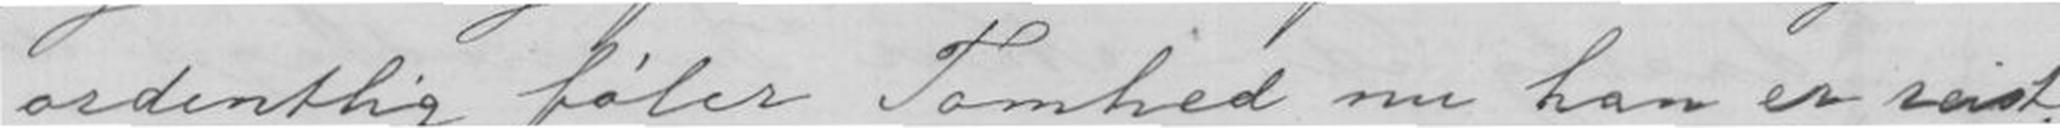ordentlig føler Tomhed nu han er reist;
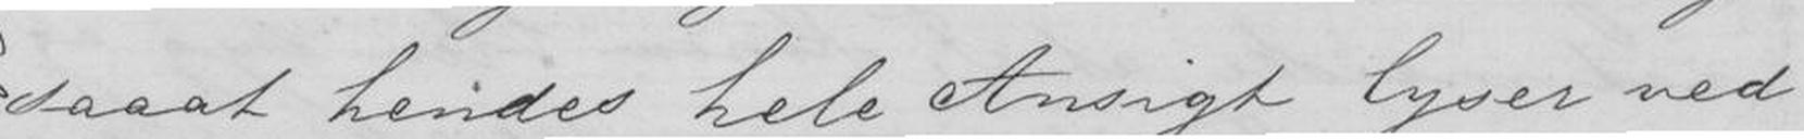saaat hendes hele Ansikt lyser ved
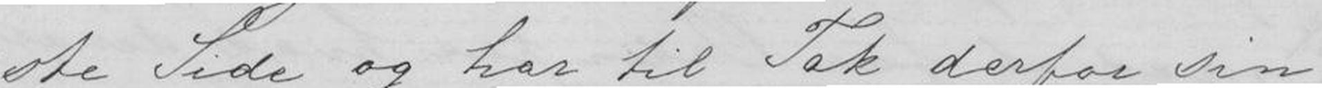ste Side og har til Tak derfor sin
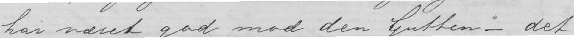har været god mod den Gutten - det
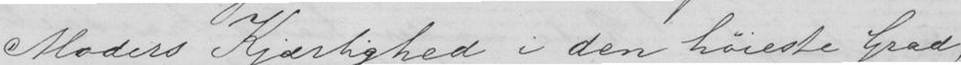Moders Kjærlighed i den høieste Grad,
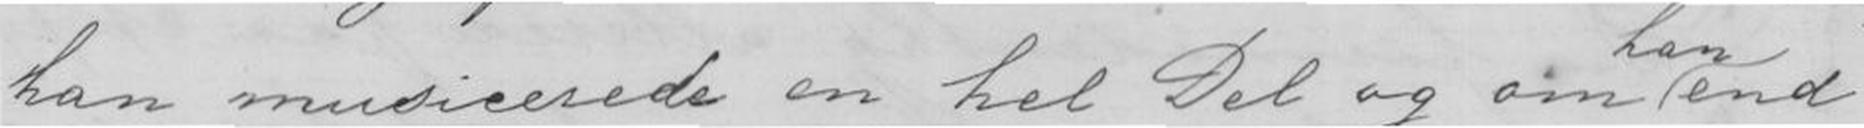han musicerede en hel Del og om end
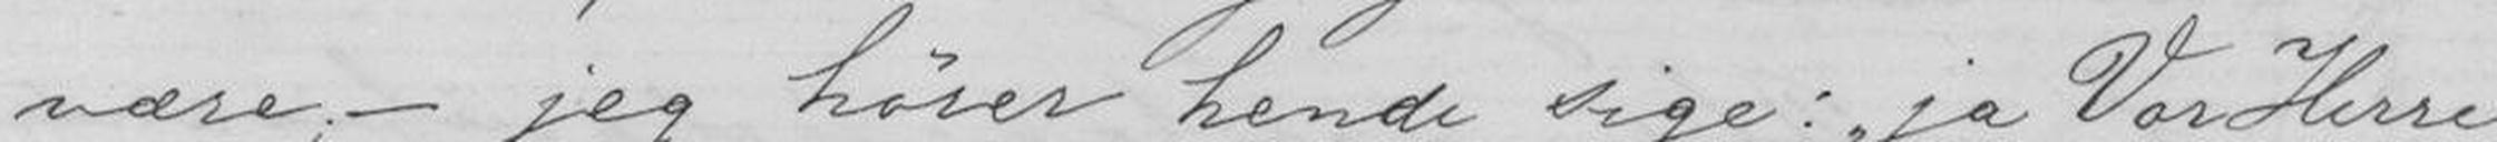være; - jeg hører hende sige: ja VorHerre
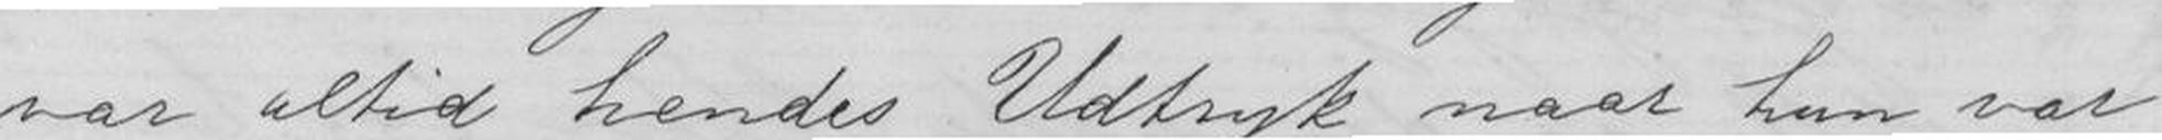var altid hendes Udtryk naar hun var
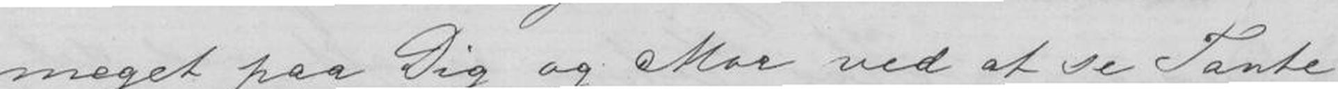meget paa Dig og Mor ved at se Tante
